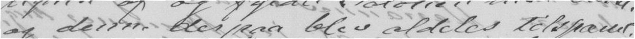og denne derpaa blev aldeles tilspærret
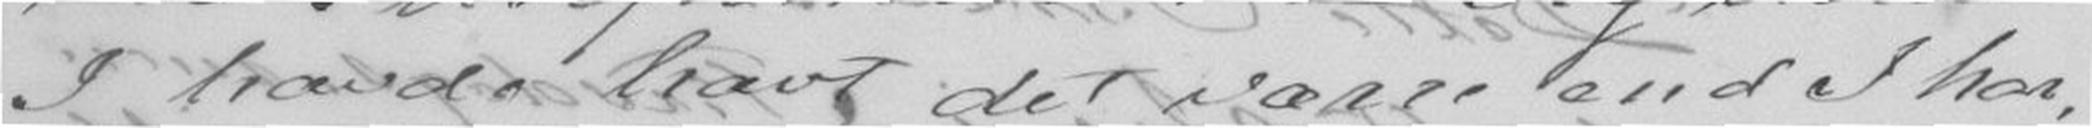I havde havt det værre end I har,
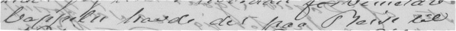Cappeln havde det paa Reise til
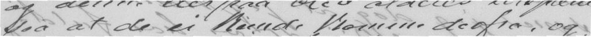saa at de ei kunde komme derfra, og
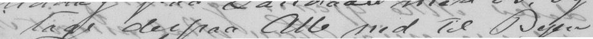tage derpaa Alle med til Byen
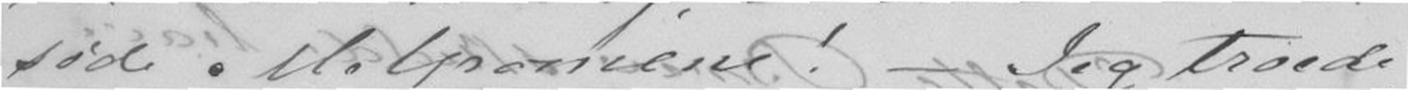søde Melponene! - Jeg troed
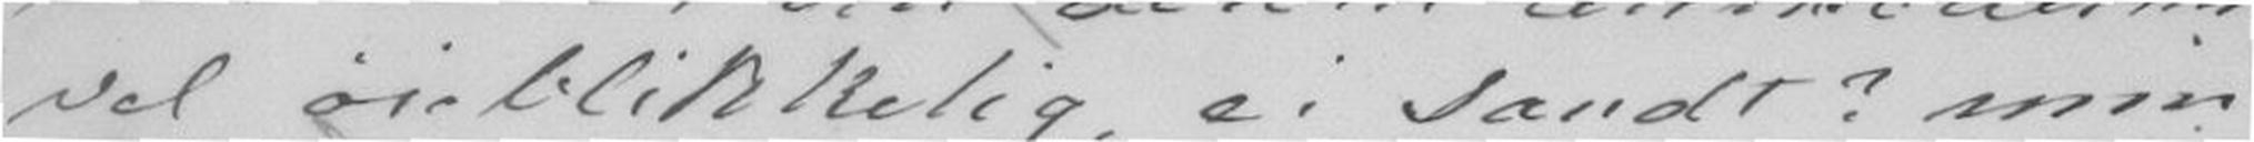vel øieblikkelig ei sandt? min
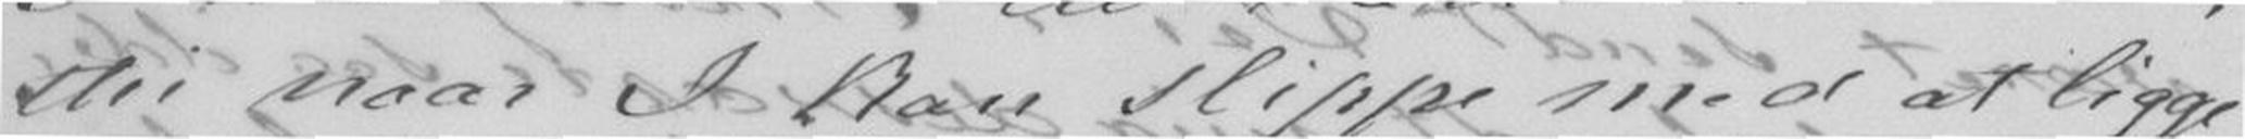thi naar I kan slippe med at ligge
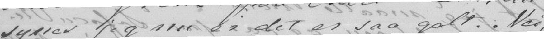synes jeg nu ei det er saa galt. Nei
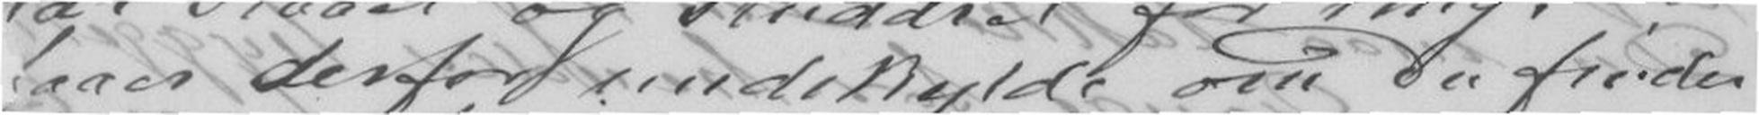aaer derfor undskylde om Du finder
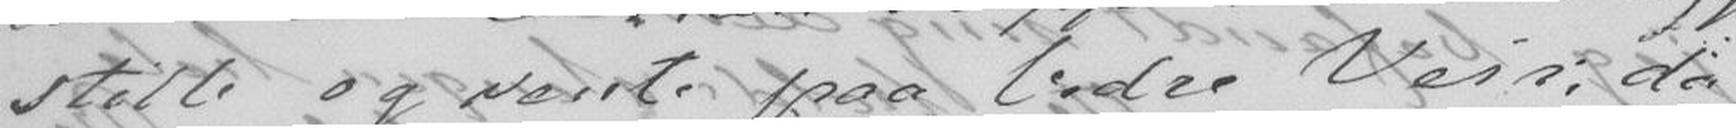stille og vente paa bedre veir, da
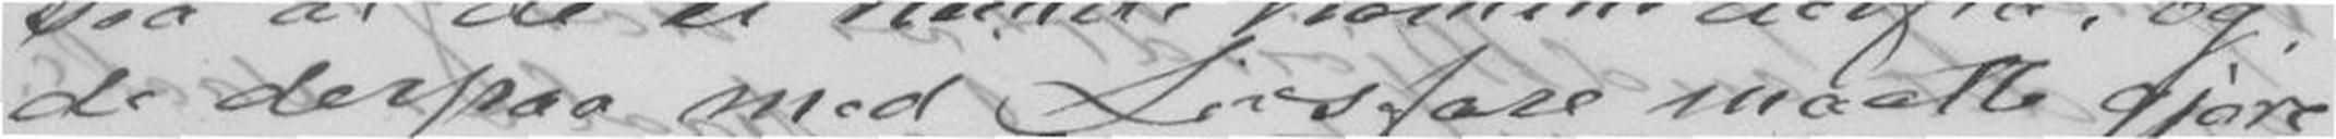de derpaa med Livsfare maatte gjøre
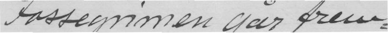Fossegrimen går frem-
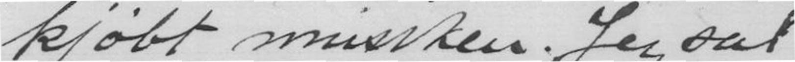kjøbt musiken. Jeg sat
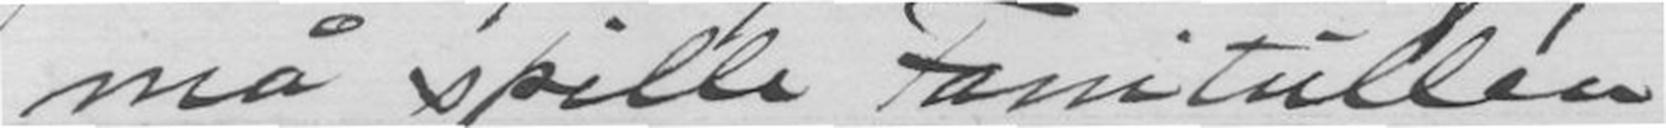må spille Fanitullen
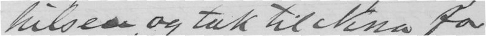hilsen og tak til Nina for
